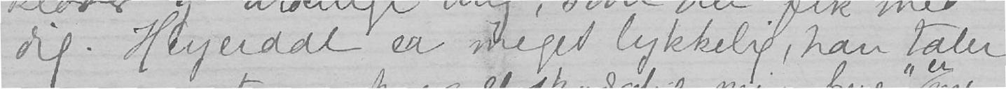dig. Heyerdal er meget lykkelig, han taler
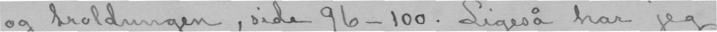og troldungen, side 96-100. Ligeså har jeg
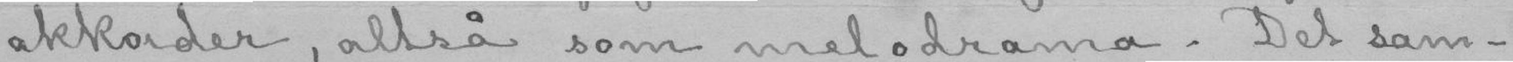akkorder, altså som melodrama. Det sam-
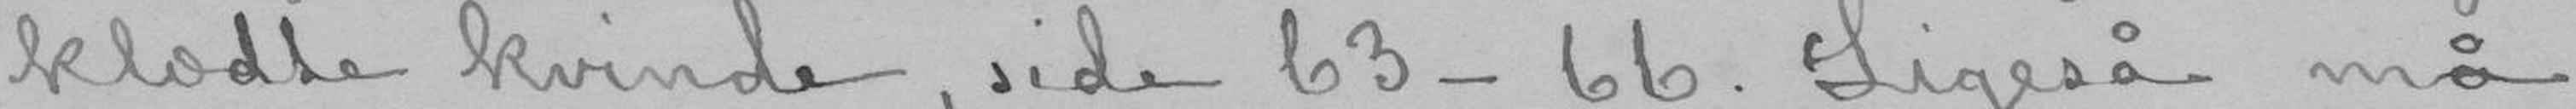klædde kvinde, side 63-66. Ligeså må
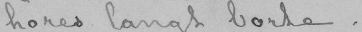høres langt borte.
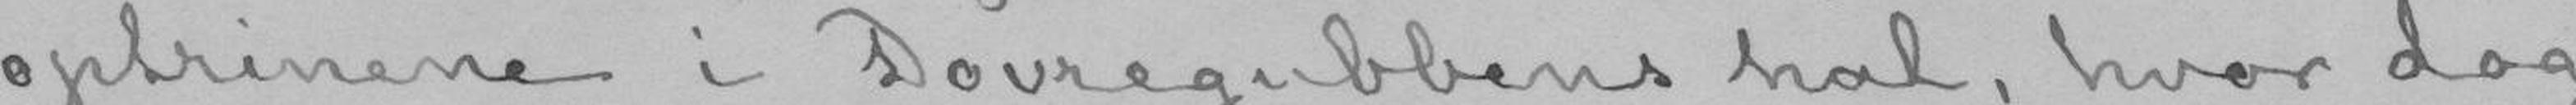optrinene i Dovregubbens hal, hvor dog
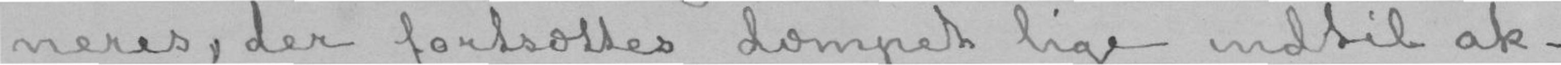neres, der fortsættes dæmpet lige indtil ak-
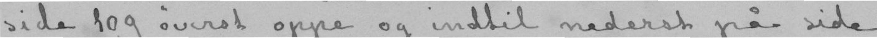side 109 øverst oppe og intil nederst på side
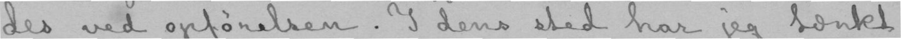des ved opførelsen. I dens sted har jeg tænkt
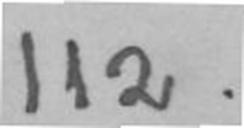112.
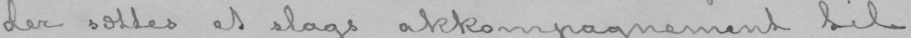der sættes et slags akkompagnement til
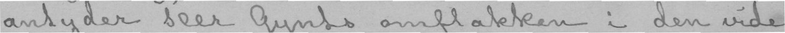antyder Peer Gynts omflakken i den vide
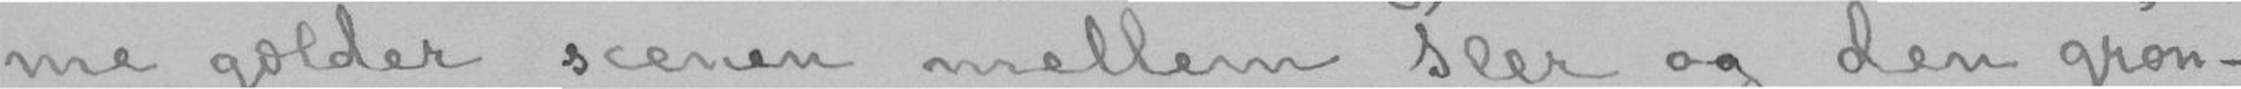me gælder scenen mellem Peer og den grøn-
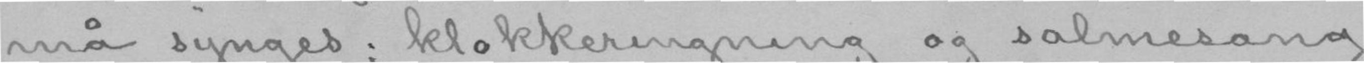må synges; klokkeringning og salmesang
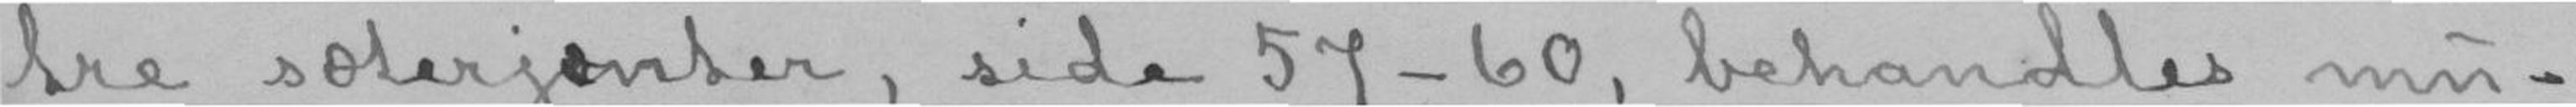tre sæterjenter, side 57-60, behandles mu-
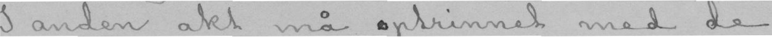I anden akt må optrinnet med de
